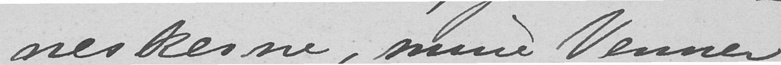neskerne, mine Venner
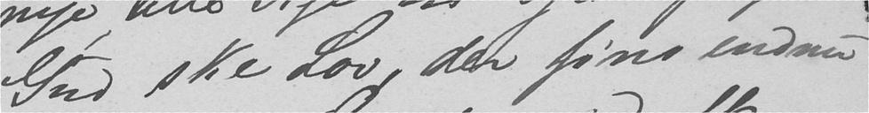Gud ske Lov, der fins endnu
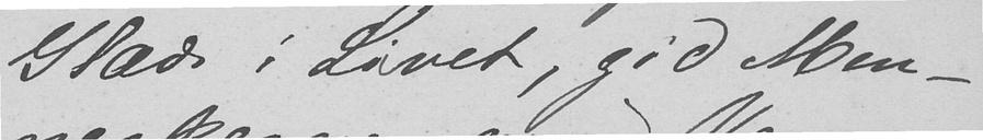Glæde i Livet, gid Men-
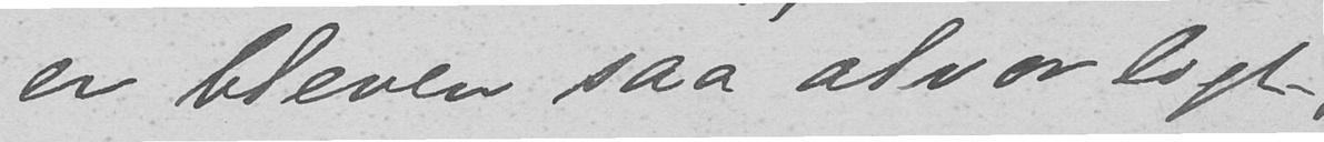er bleven saa alvorligt.
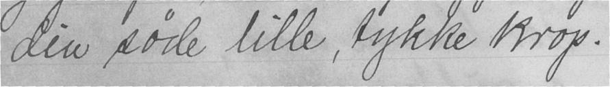din søde lille, tykke krop.
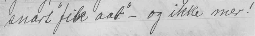snart "fibe aat" - og ikke mer!
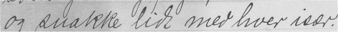og snakke lidt med hver især.
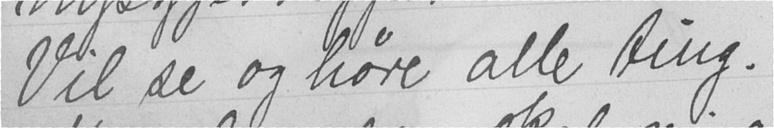Vil se og høre alle ting.
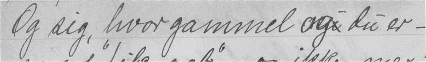Og sig, hvor gammel ogi du er -
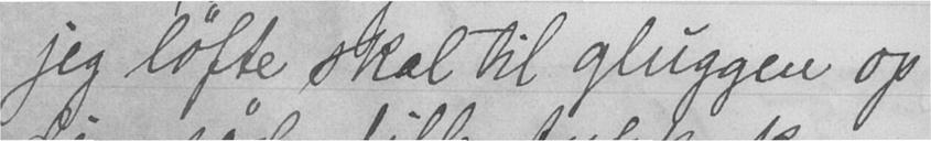jeg løfte skal til gluggen op
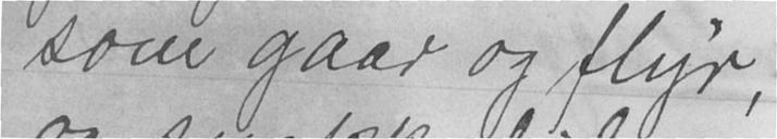som gaar og flyr,
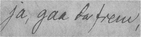Ja, gaa da frem,
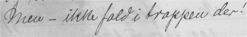Men - ikke fald i trappen der!
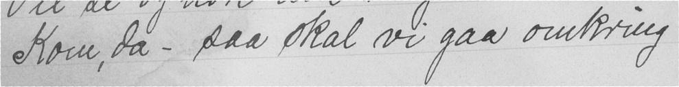Kom, da - saa skal vi gaa omkring
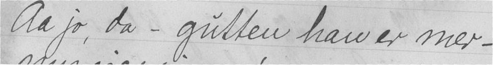Aa jo, da - gutten han er mer -
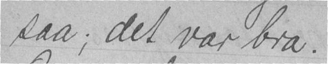saa; det var bra.
